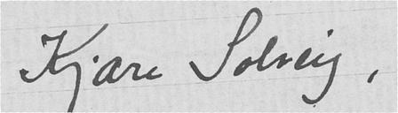Kjære Solveig,
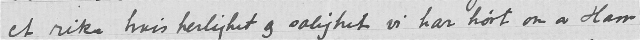et rike hvis herlighet og salighet vi har hørt om av Ham
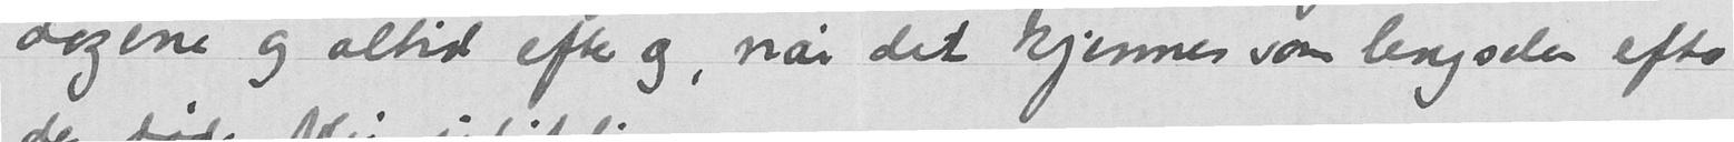dagene og altid efter og, når det kjennes som lengselen efter
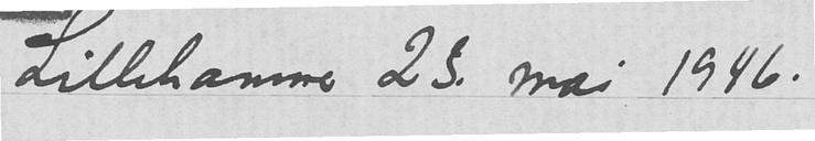Lillehammer 23. mai 1946.
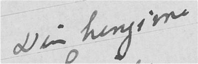Din hengivne
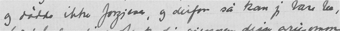og dødde ikke forgjeves, og derfor så kan jeg bare be,
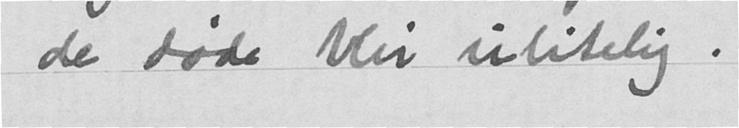de døde blir ulidelig.
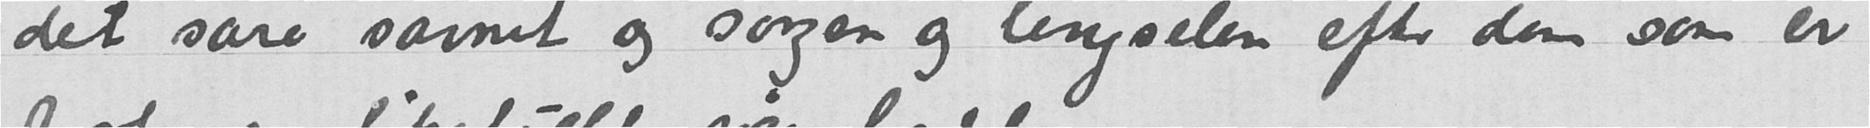det såre savnet og sorgen og lengselen efter dem som er
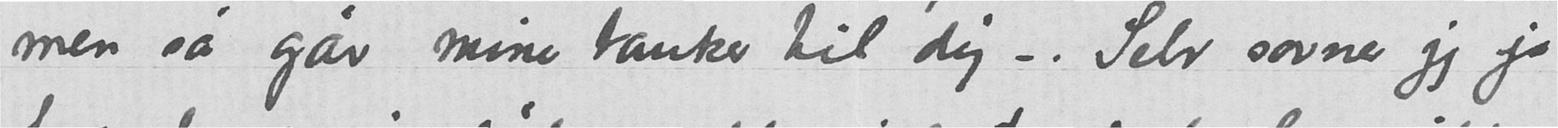men så går mine tanker til dig –. Selv savner jeg jo
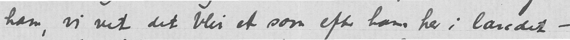ham, vi vet det blir et savn efter ham her i landet –
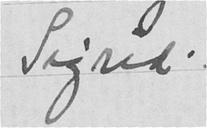Sigrid.
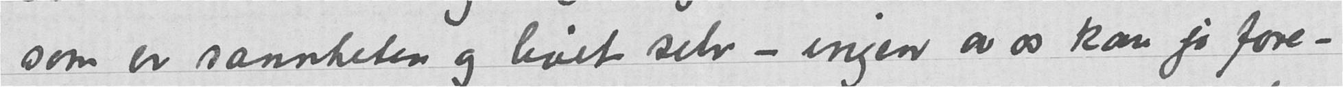som er sannheten og livet selv - ingen av os kan jo fore-
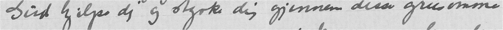Gud hjelpe dig og styrke dig gjennem disse grusomme
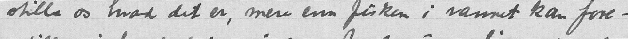stille os hvad det er, mere enn fisken i vannet kan fore-
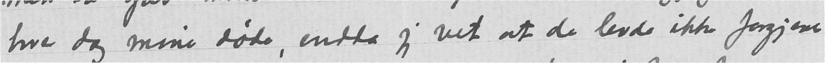hver dag mine døde, endda jeg vet at de levde ikke forgjeves
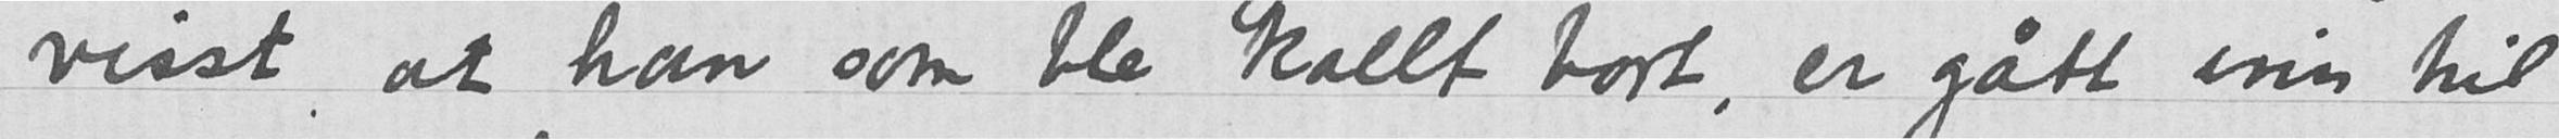visst at han som ble kallt bort, er gått inn til
